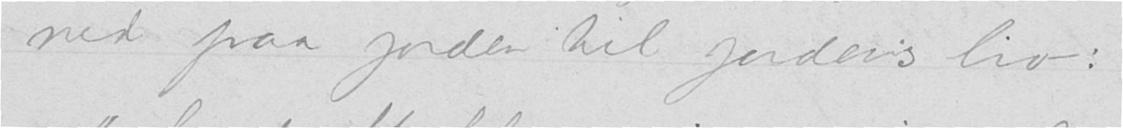ned paa jorden til jordens liv:
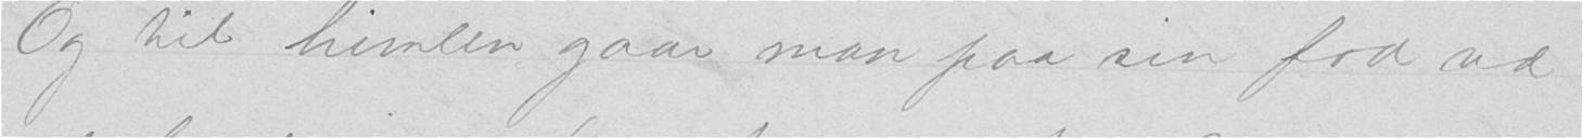Og til himlen gaar man paa sin fod ud
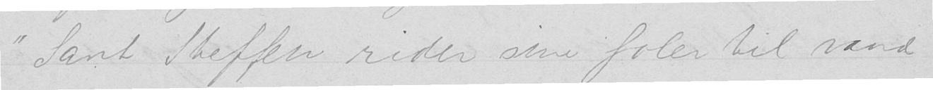"Sant Steffen rider sine foler til vand
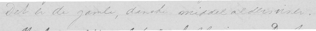Det er de gamle, danske middelalderviser.
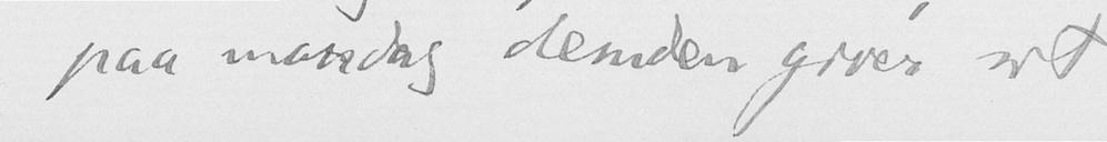paa mandag desuden giver sit
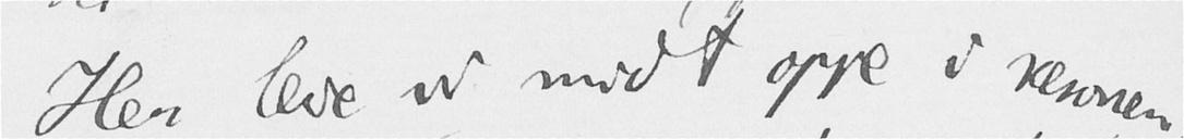Her leve vi midt oppe i kennen,
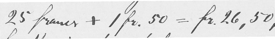25 francs + 1 fr. 50 = fr. 26,50,
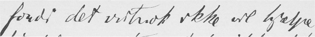fordi det vistnok ikke vil hjælpe
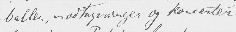baller, modtagninger og koncerter
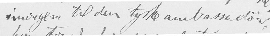imorgen til den tyske ambassadør,
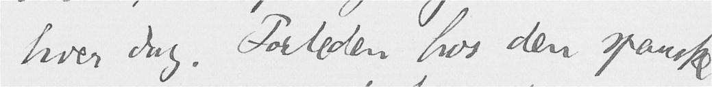hver dag. Forleden hos den spanske,
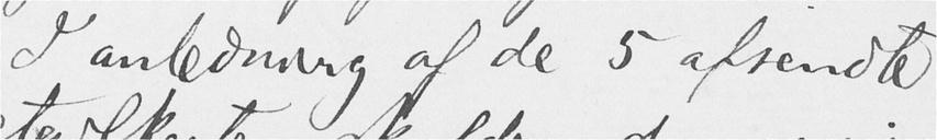I anledning af de 5 afsendte
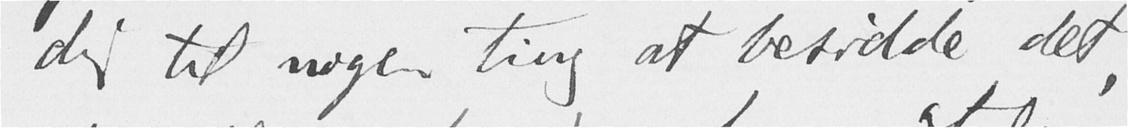dig til nogen ting at besidde det,
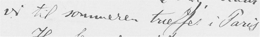vi til sommeren træffes i Paris.
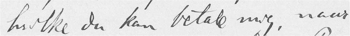hvilke du kan betale mig, naar
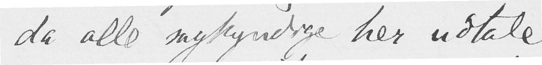da alle sagkyndige her udtale
